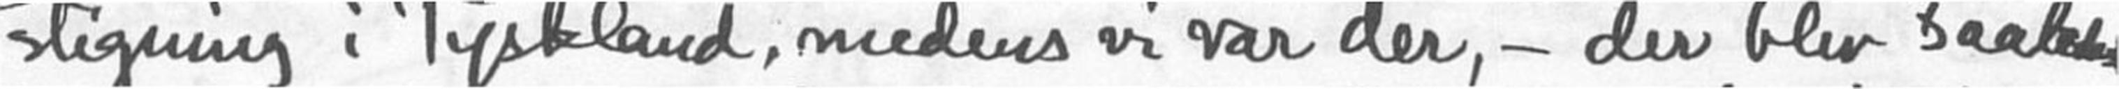stigning i Tyskland, medens vi var der,- der blev saaledes
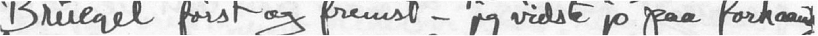Bruegel først og fremst - jeg vidste jo paa forhaand
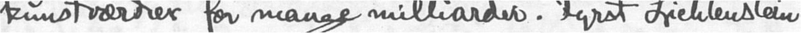kunstværdier for mange milliarder. Fyrst Liechtenstein
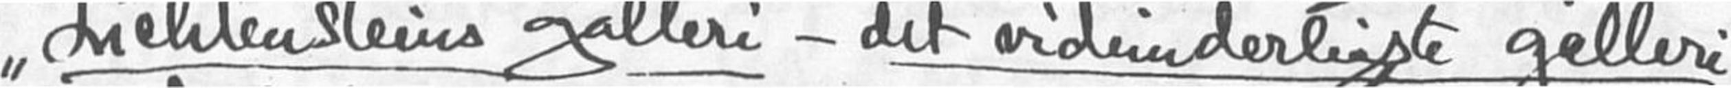i "Liechtensteins galleri" - det vidunderligste galleri
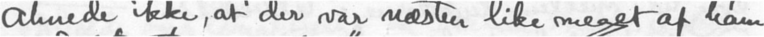ahnede ikke, at der var næsten like meget af ham
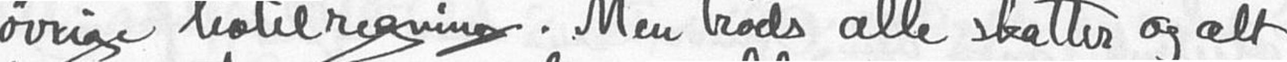øvrige hotelregning. Men trods alle skatter og alt
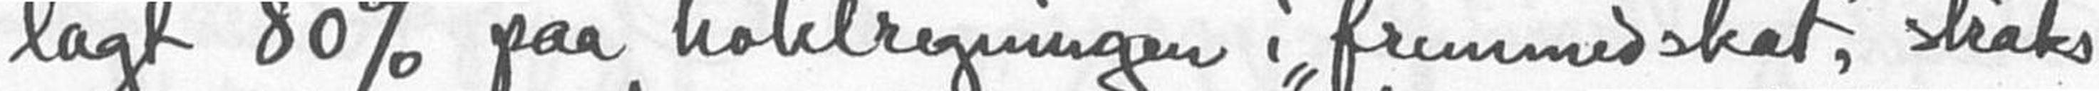lagt 80% paa hotelregningen i "fremmedskat", strax
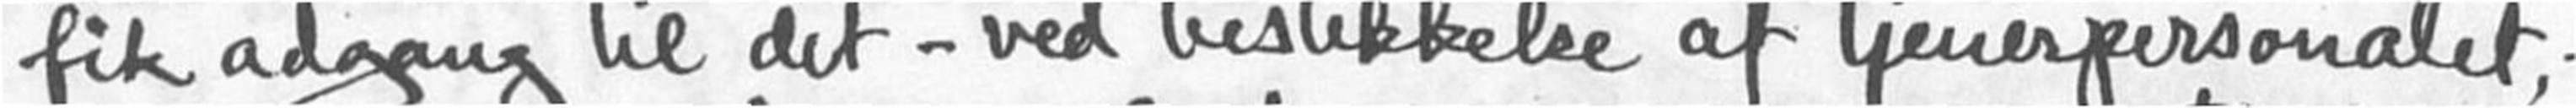fik adgang til det - ved bestikkelse av tjenerpersonalet, -
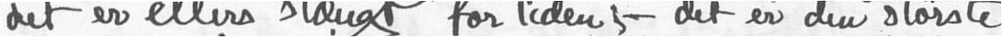det er ellers stængt for tiden, - det er den største
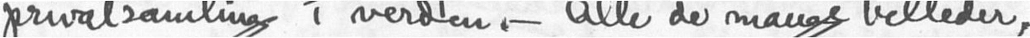privatsamling i verden. - Alle de mange billeder
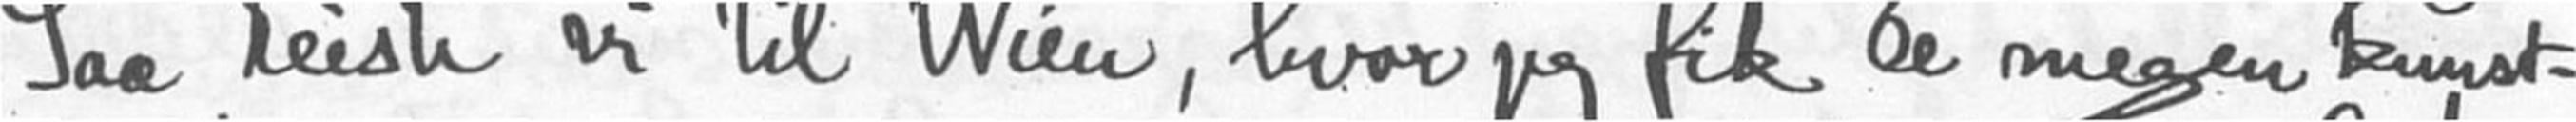Saa reiste vi til Wien, hvor jeg fik se megen kunst -
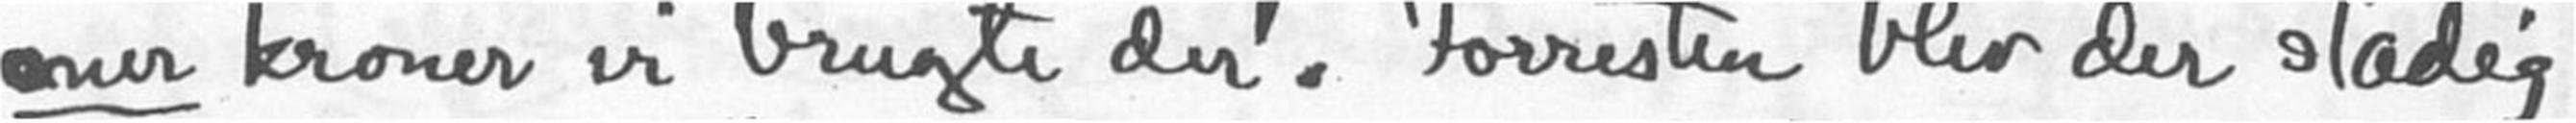oner kroner vi brugte der. Forresten blev der stadig
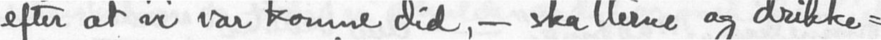efter at vi var komne did, - skatterne og drikke-
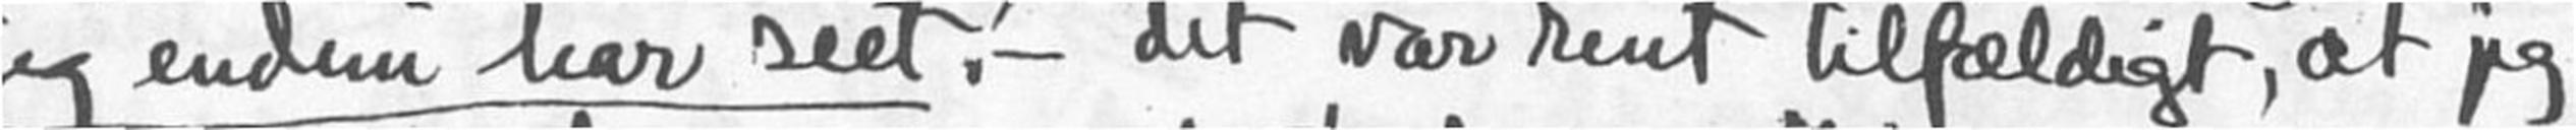jeg endnu har seet! - det var rent tilfældigt, at jeg
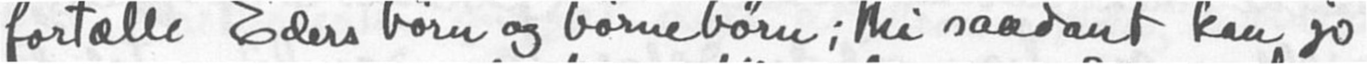fortælle Eders børn og børnebørn ; thi saadant kan jo
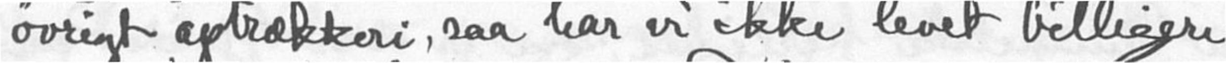øvrigt optrækkeri, saa har vi ikke levet billigere
